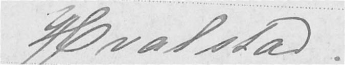Hvalstad
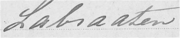Labraaten
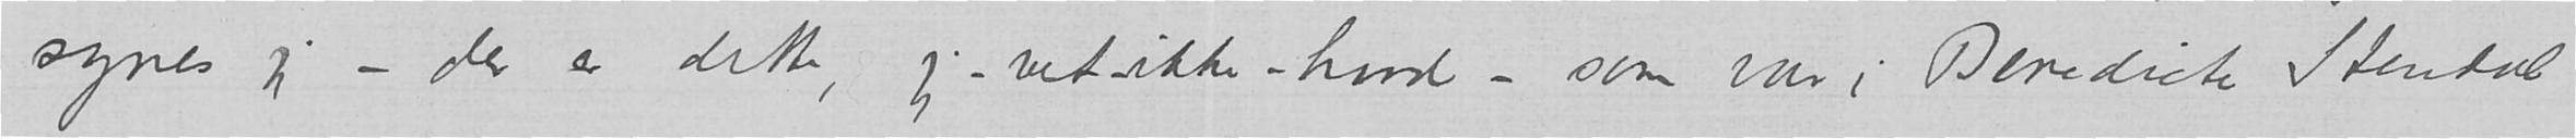synes jeg – der er dette, jeg-vet-ikke-hvad-som var i Benediete Stendal
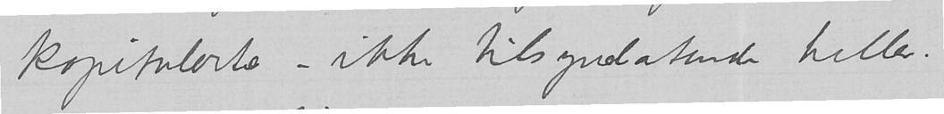kapitulerte – ikke tilsynlatende heller.
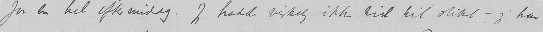for en hel eftermiddag. Jeg hadde virkelig ikke tid til slikt – jeg har
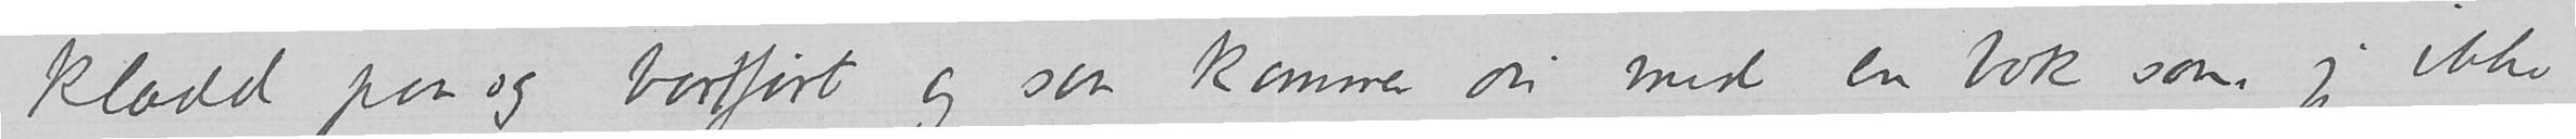klædd paa og bortført og saa kommer du med en bok som jeg ikke
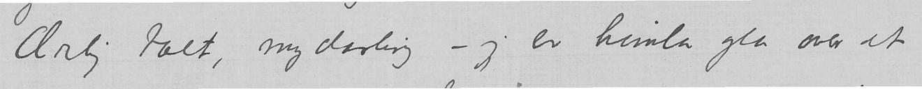Ærlig talt, my darling – jeg er himla gla over at
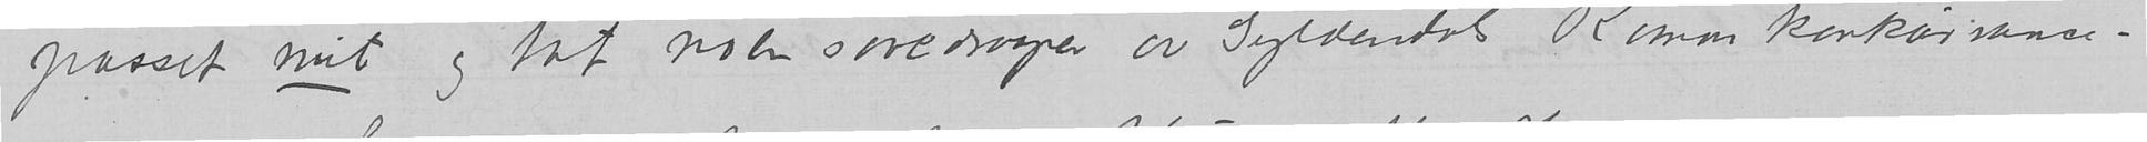passet mit og tat noen sovedraaper av Gyldendals Romankonkurrance-
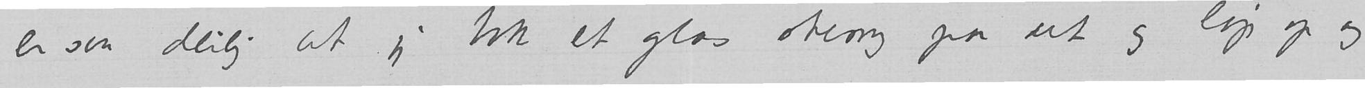er saa deilig at jeg tok et glas sherry paa ut og løp op og
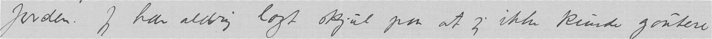for den. Jeg har aldrig lagt skjul paa at jeg ikke kunde goutere
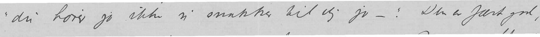«du hører jo ikke vi snakker til dig jo –.» Den er fært god,
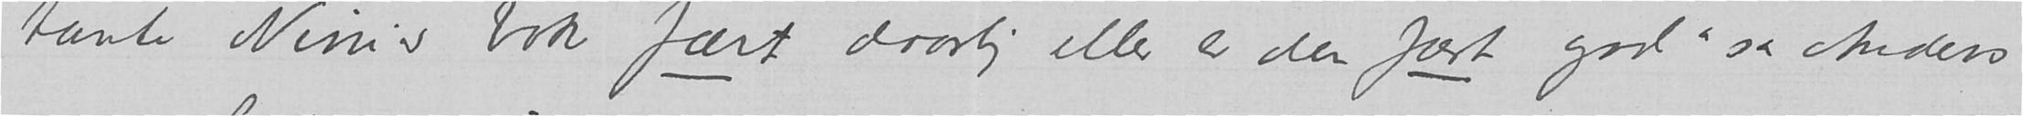tante Ninis bok fært daarlig eller er den fært god» sa Anders
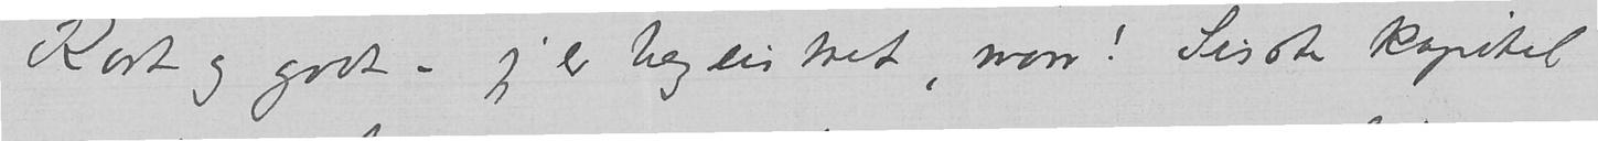Kort og godt – jeg er begeistret, ven! Sisste kapitel
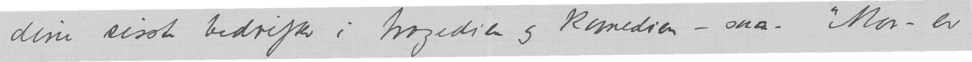dine sisste bedrifter i tragedien og komedien – saa – «Mor – er
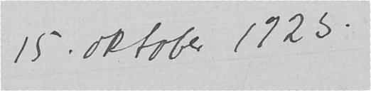15. oktober 1923.
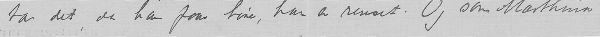tar det, da han faar høre, han er renset. Og som Marthina
